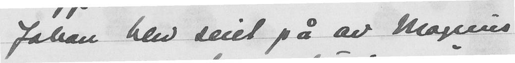Johan blev reiet på av Magnus
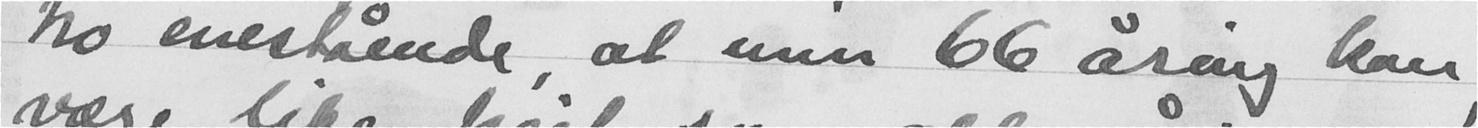No enestående, at min 66 åring kan
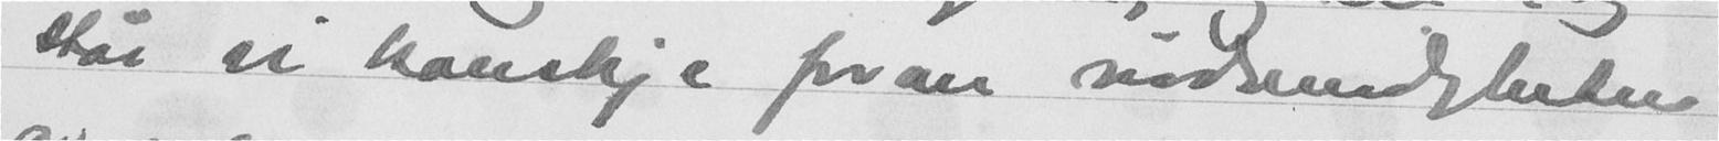står vi kanskje foran nødvendigheten
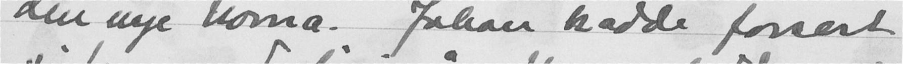den nye Norna. Johan hadde forsert
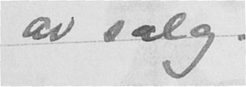av salg.
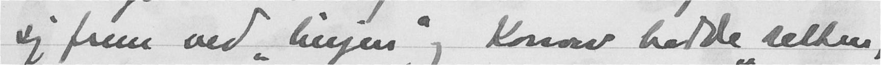sig frem ved "linjen og Konow hadde selten;
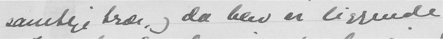samtlige trær, og da blev vi liggende
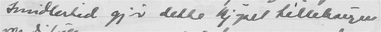Imidlerted gjør dette kjøpet lillehaugen
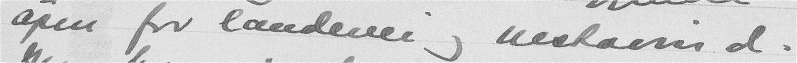åpen for landevei og vestavind.
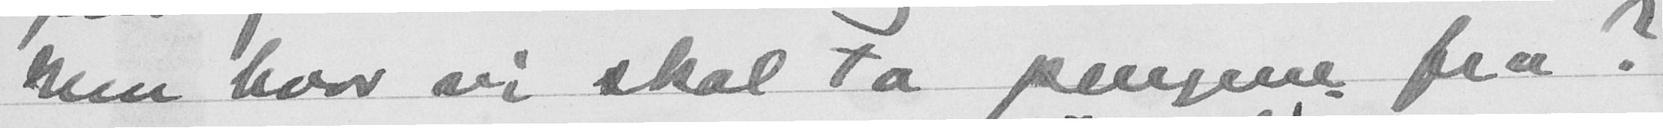Men hvor vi skal ta pengene fra?
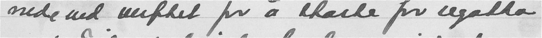nede ved verftet for å starte for regatta
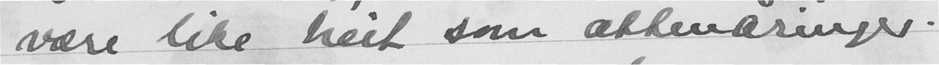være like heit som attenåringer.
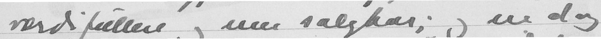værdifullere og mer salgbar; og en dag
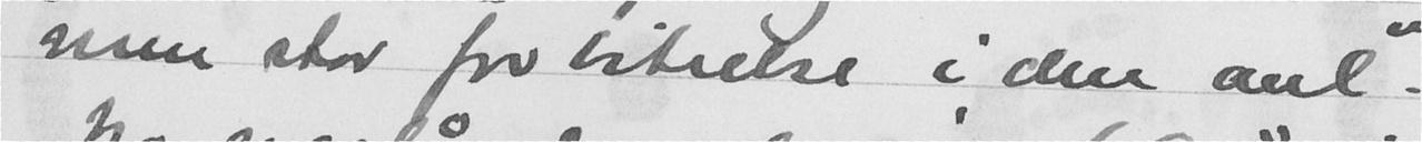men stor forbitrelse i den anl."
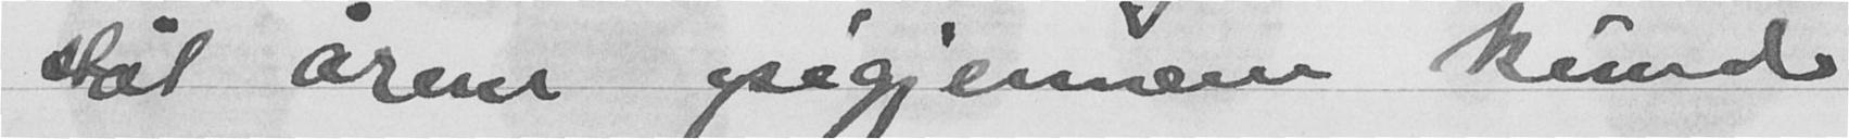støt årene opigjennom kunde
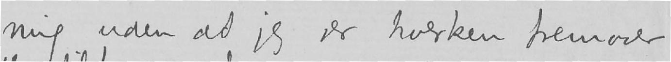mig uden at jeg ser hverken fremover
